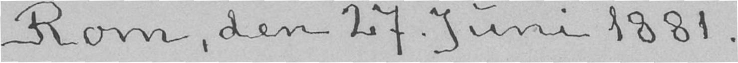Rom, den 27. Juni 1881.
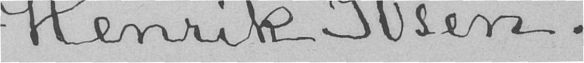Henrik Ibsen.
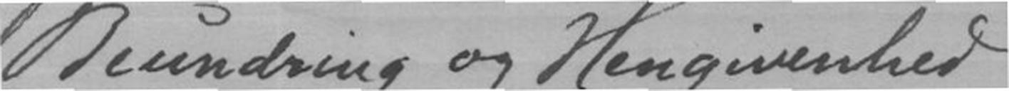Beundring og Hengivenhed
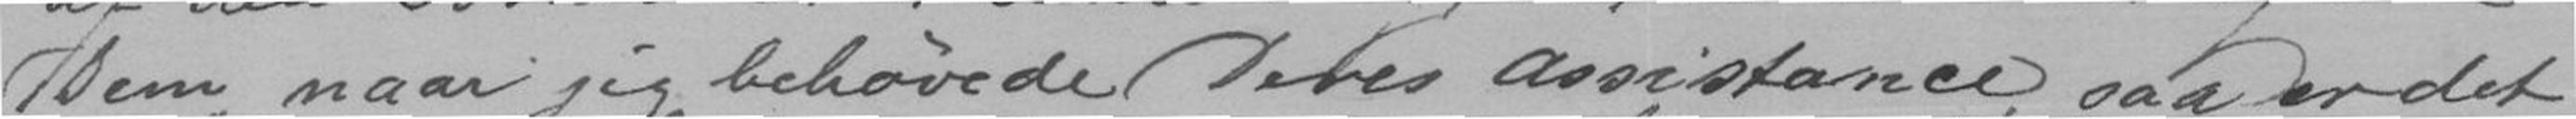Dem, naar jeg behøvede Deres Assistance saa er det
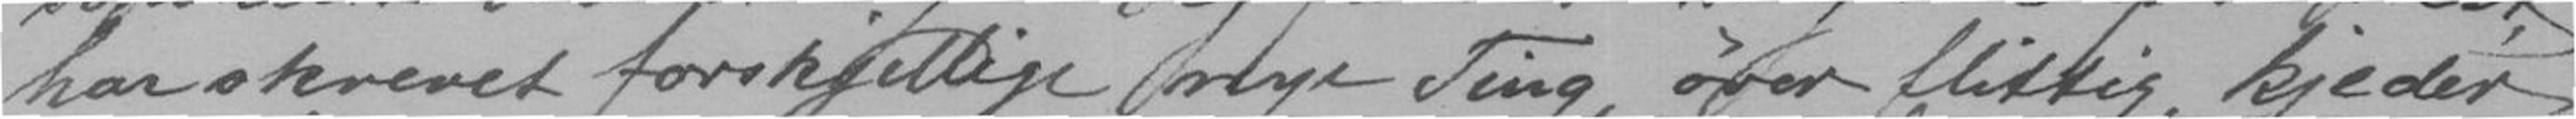har skrevet forskjellige nye Ting, øver flittig, kjeder
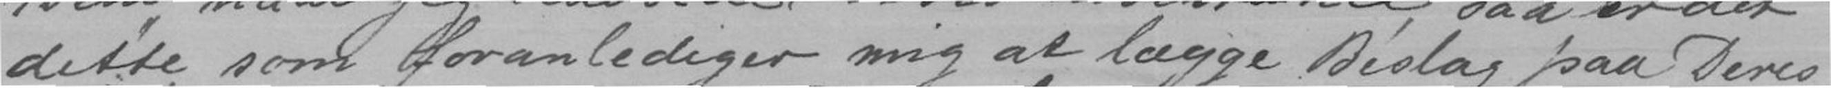dette, som foranlediger mig at lægge Béslag paa Deres
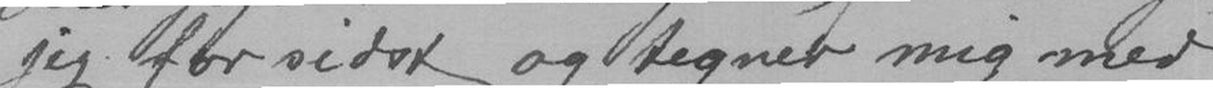jeg for sidst og tegner mig med
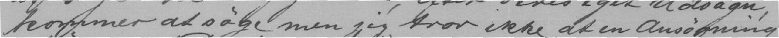kommer at søge, men jeg tror ikke at en Ansøgning,
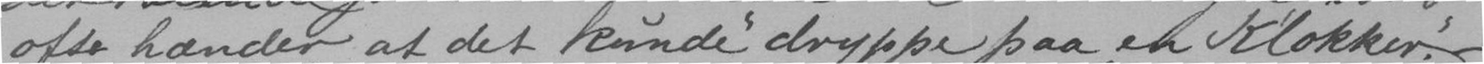ofte hænder, at det kunde "dryppe paa en Klokker". -
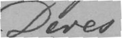Deres
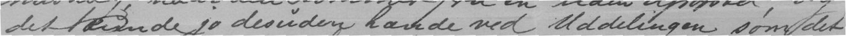det Kunde jo desuden hænde ved Uddelingen, som det
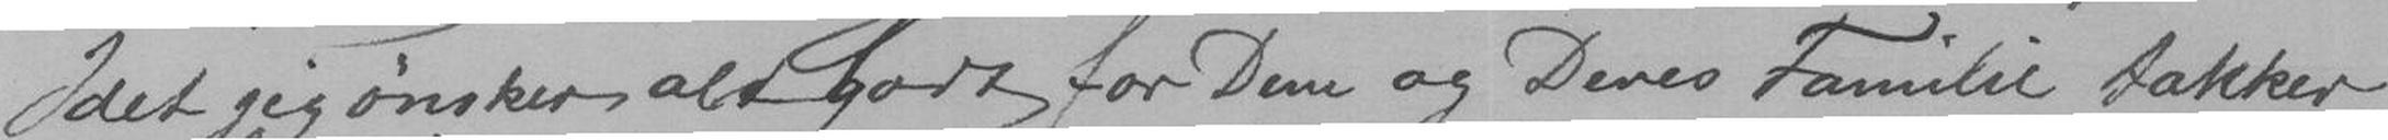Idet jeg ønsker alt Godt for Dem og Deres Familie takker
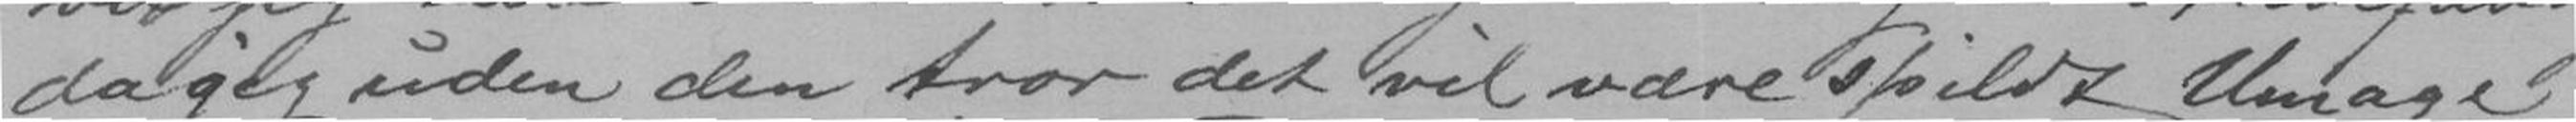da jeg uden den tror det vil være spildt Umage
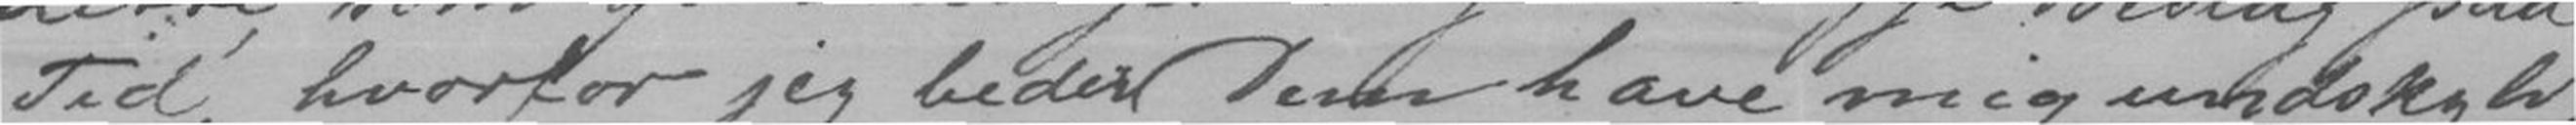Tid, hvorfor jeg beder Dem have mig undskyldt.
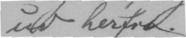ud herfra.
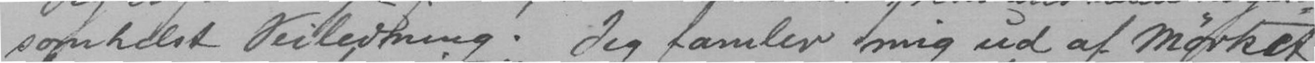somhelst Veiledning. Jeg famler mig ud af Mørket
